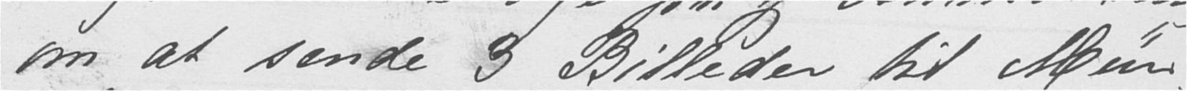om at sende 3 Billeder til Mün-
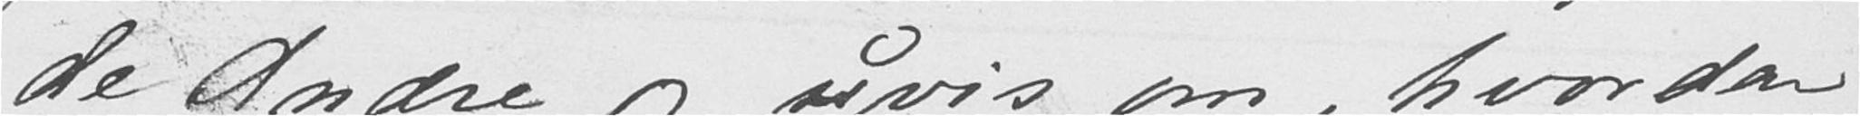de Andre og uvis om, hvordan
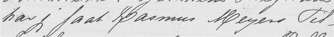har jeg faat Rasmus Meyers Til-
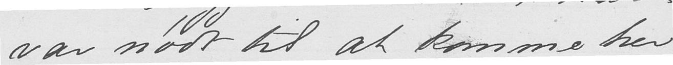var nødt til at komme her-
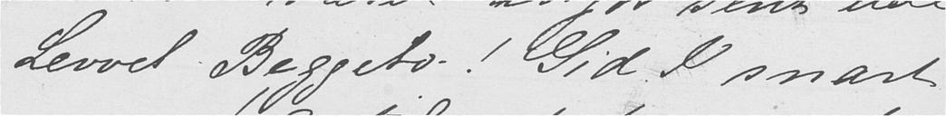Levvel Beggeto! Gid I snart
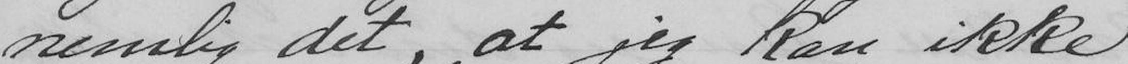nemlig det, at jeg kan ikke
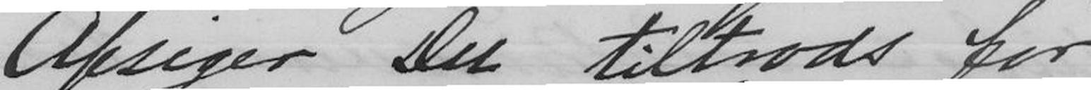Afsiger Du tiltrods for
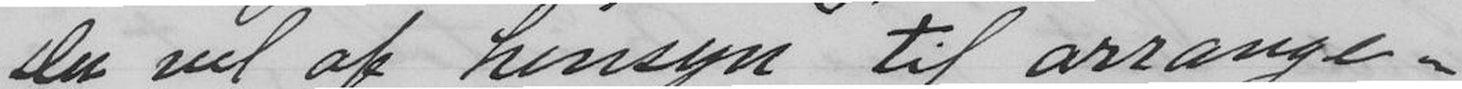Du vel af hensyn til arrange -
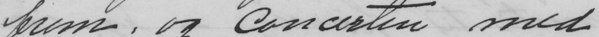frem, og Concerten med
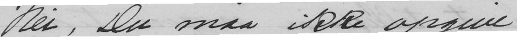Nei, du maa ikke opgive
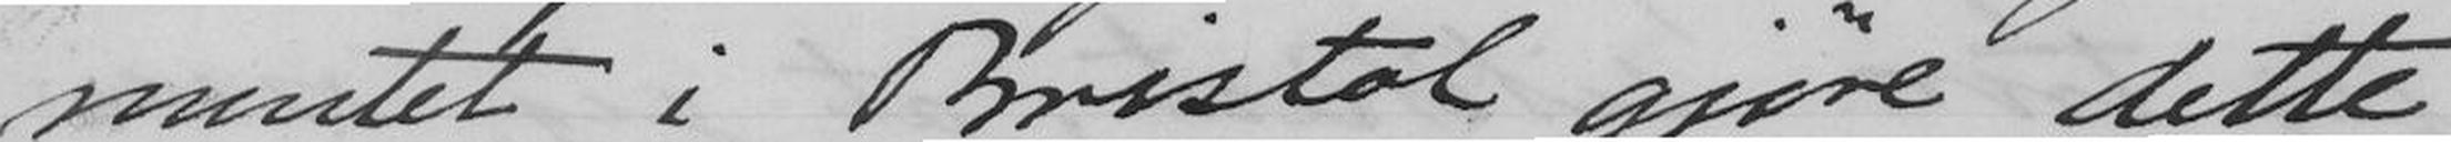mentet i Bristol gjøre dette
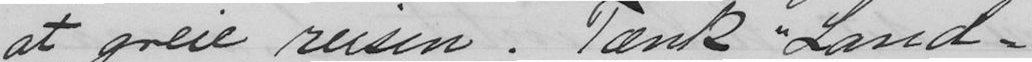at greie reisen. Tænk "Land-
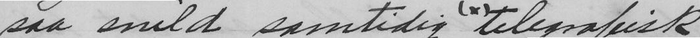saa snild samtidig telegrafisk
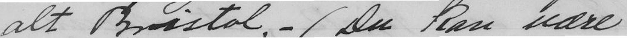alt Bristol, - (Du kan være
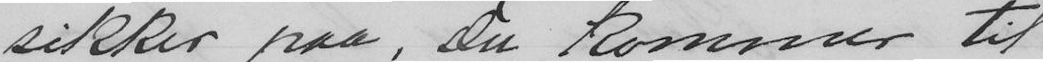sikker paa, Du kommer til
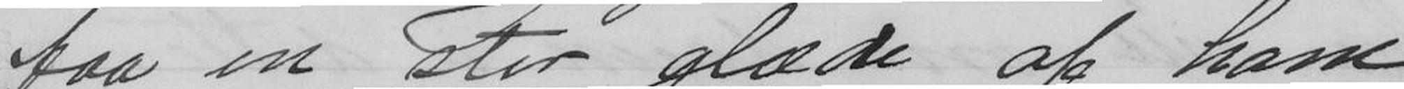faa en stor glæde af ham.
